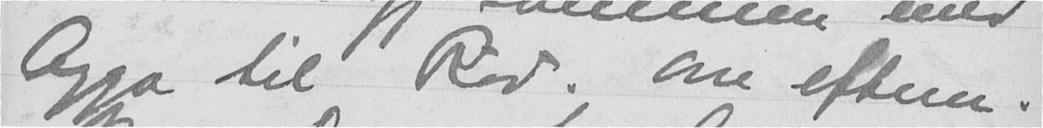Agga til Rød. Om efterm.
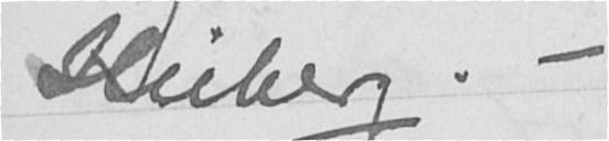Heiberg. -
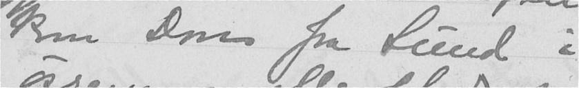kom Don fra Sund i
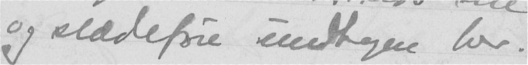og slædeføre undtagen her.
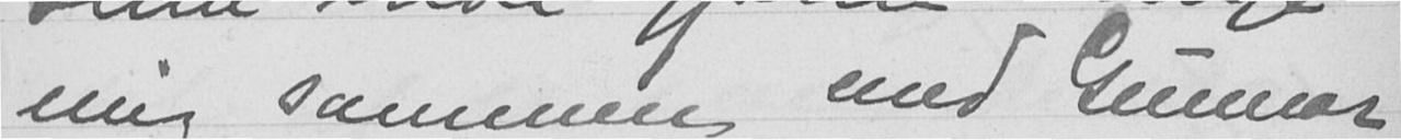mig sammen med Gunnar
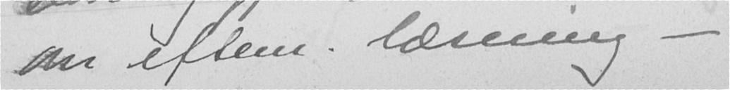Om efterm. læsning -
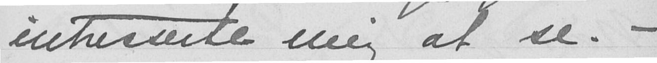intresserte mig at se. -
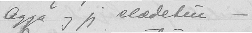Agga og jeg slædetur -
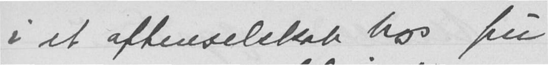i et aftenselskab hos fru
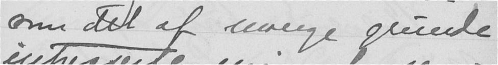som det af mange grunde
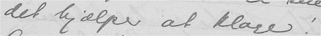det hjælper at klage!
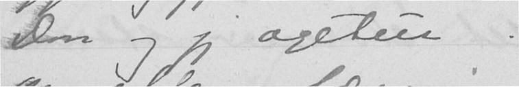Don og jeg agetur.
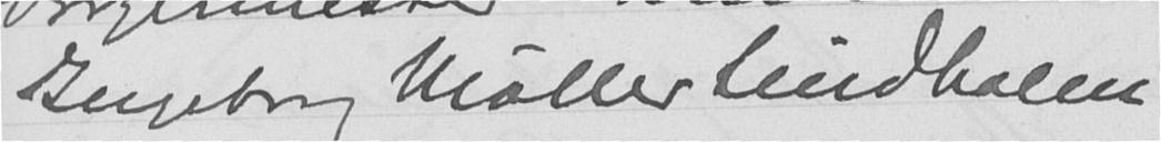Ingeborg Møller Lindholm
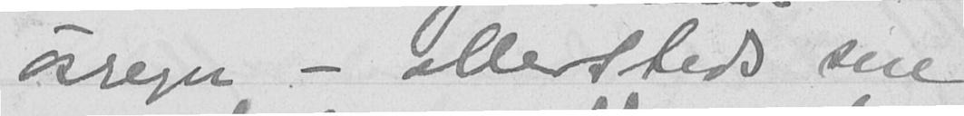øsregn - allesteds sne
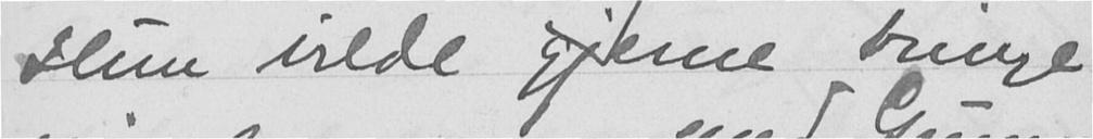Hun vilde gjerne bringe
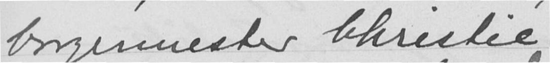borgermester Christie
